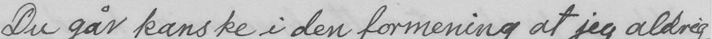Du går kanske i den formening at jeg aldrig
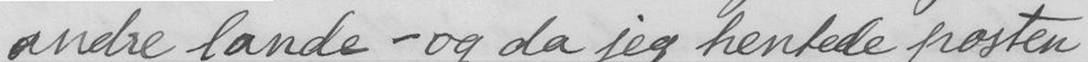andre lande - og da jeg hentede posten
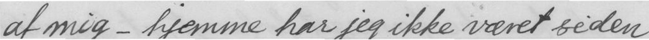af mig - hjemme har jeg ikke været siden
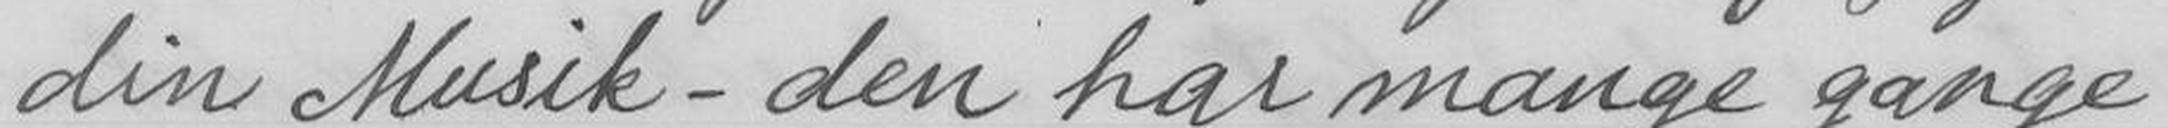din Musik - den har mange gange
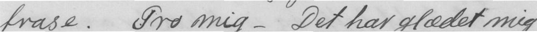frase. Tro mig - Det har glædet mig
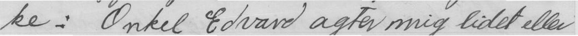ke: Onkel Edvard agter mig lidet eller
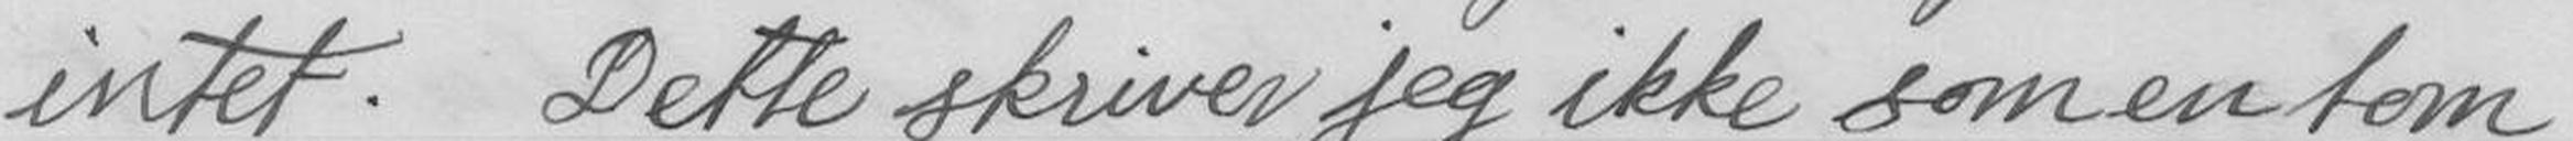intet. Dette skriver jeg ikke som en tom
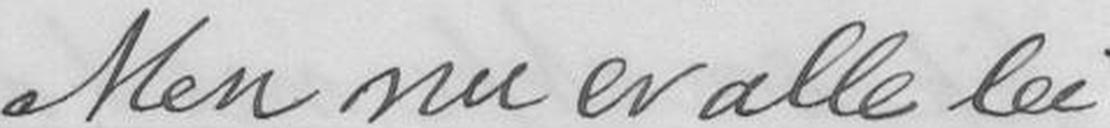Men nu er alle lei
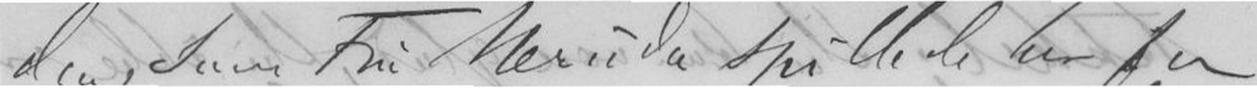den, som Fru Neruda spillede her for
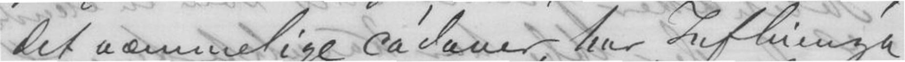det væmmelige Cadaver, har Influenza
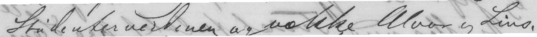Studenterverden og vække Alvor og Livs-
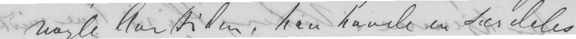nogle Aar siden, han havde en særdeles
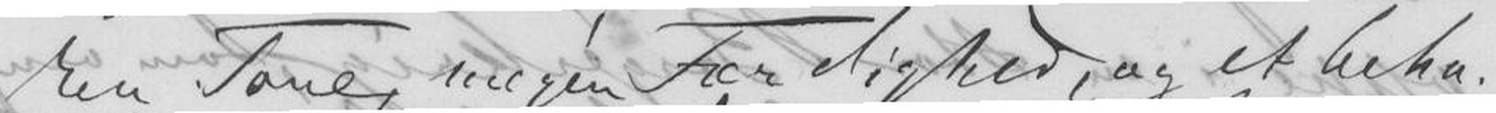ren Tone, megen Færdighed, og et beha-
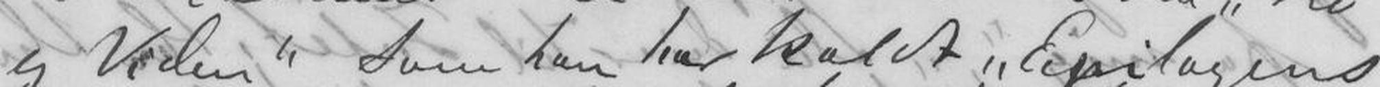og Veden, som han har kaldt Epilagens
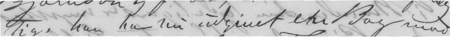tig; han har nu udgivet en Bog mod
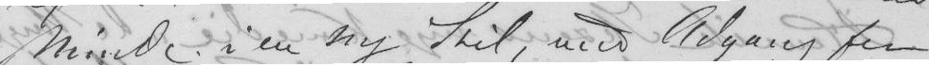Minde i en ny Stil, med Adgang for
